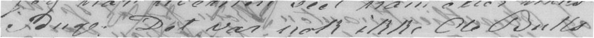uge. Det var nok ikke Ole Bulls
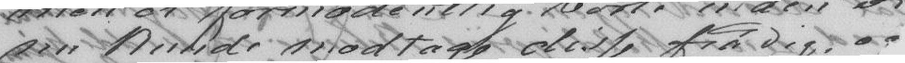nu kunde modtage disse fra Dig, og
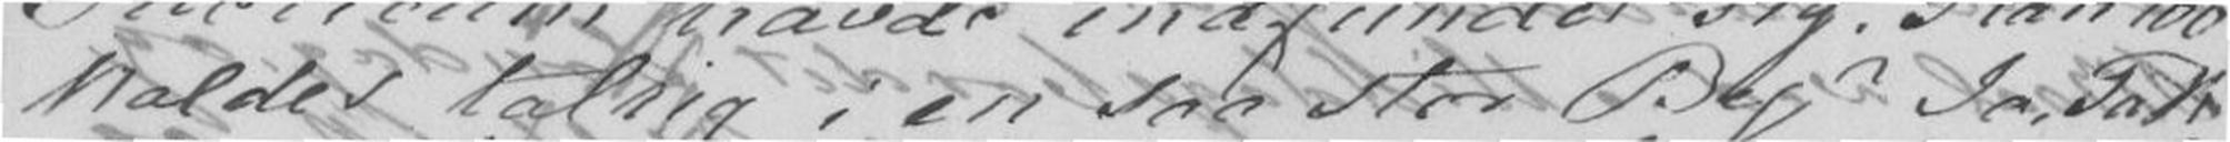kaldes talrig i en stor By? Ja, Tak
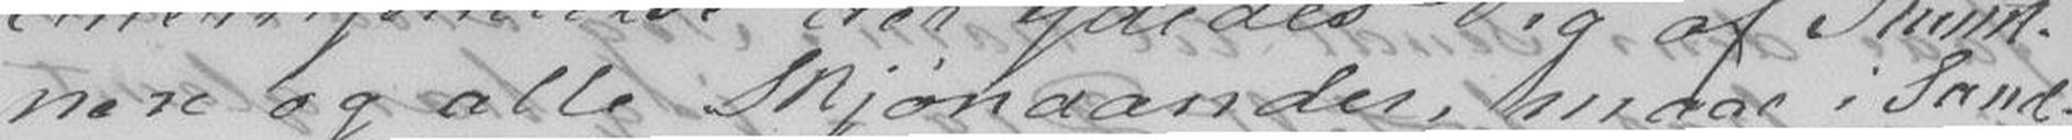nere og alle Skjønaander, maae i Sand-
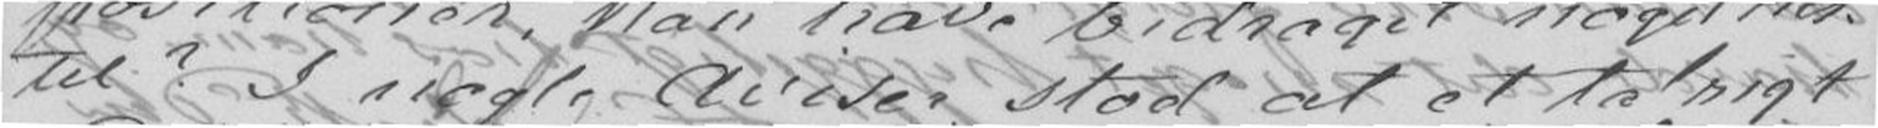til? I nogle Aviser stod at et talrigt
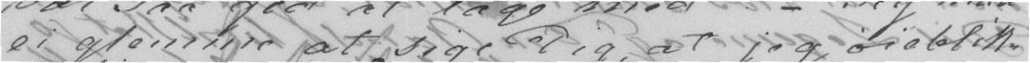ei glemme at sige Dig, at jeg øieblik-
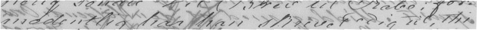modentlig har han skrevet dig til, thi
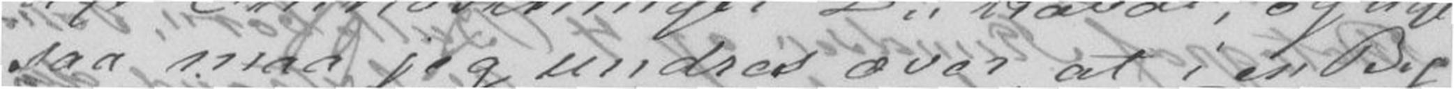saa maa jeg undres over at i en Bu
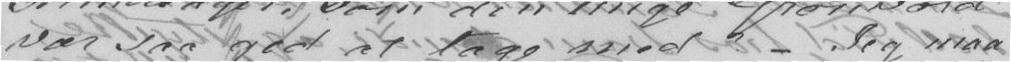var saa god at tage med. - Jeg maa
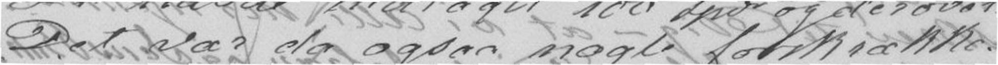Det var da ogsaa nogle forskrække-
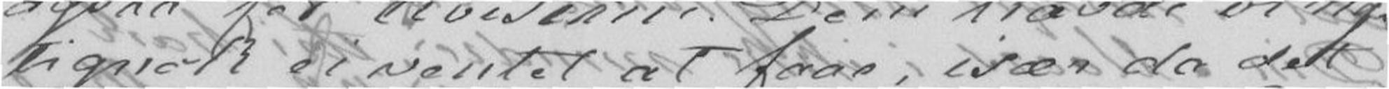tignok ei ventet at faae, især da det
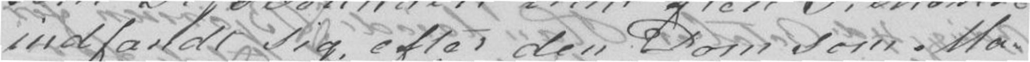indfandt sig efter den Dom som Ma-
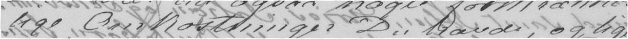lige Omkostninger Du havde, og lige-
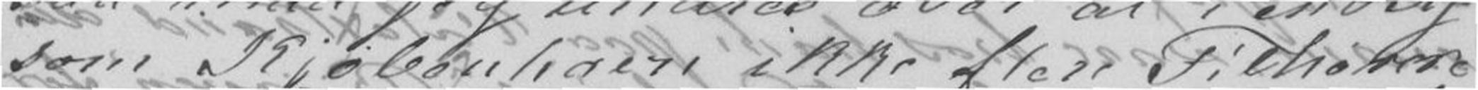som Kjøbenhavn ikke flere Tilhørere
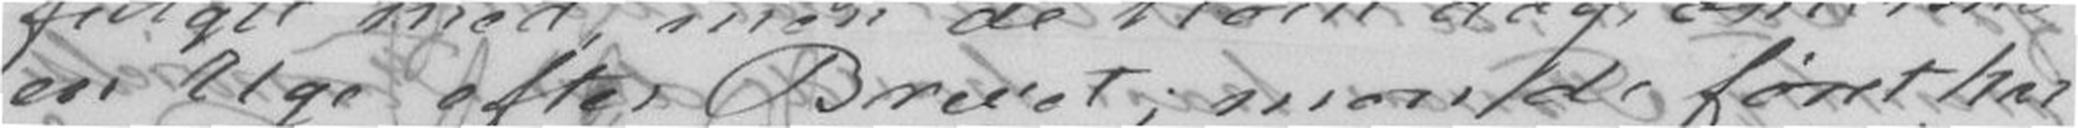en Uge efter Brevet, mon da først har
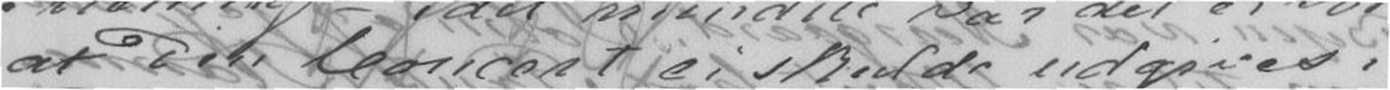at Din Concert ei skulde udgives i
